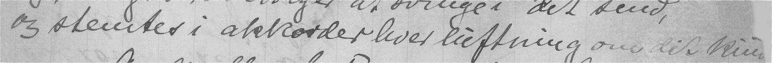og stemtes i akkorder hver luftning om dit kind.
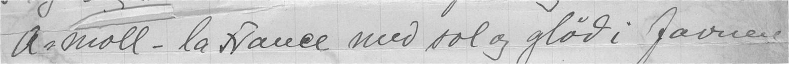A-moll - la France med sol og glød i favnen
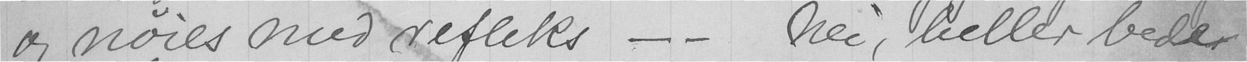og nøies med refleks - - Nei, heller beder
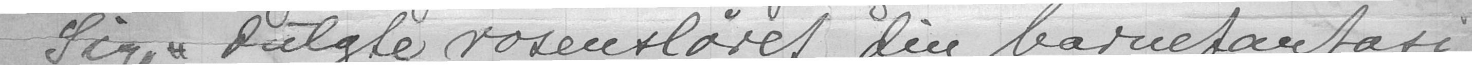Sig, dulgte rosensløret din barnefantasi,
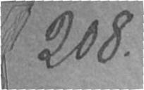208.
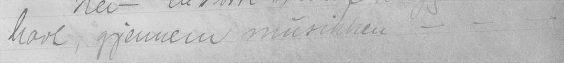havt, gjennem musikken! - - -
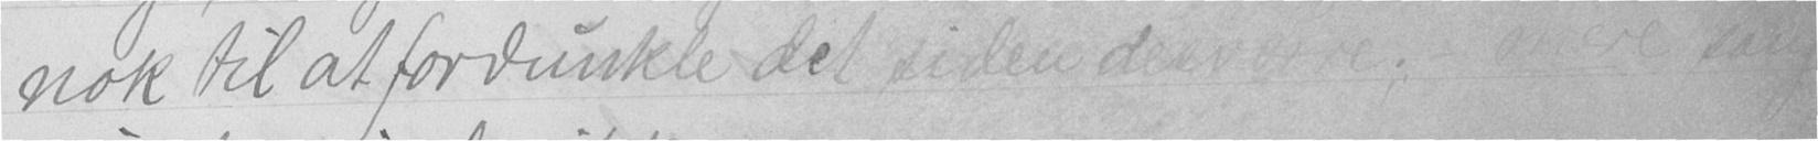nok til at fordunkle det siden desværre, mere sang-
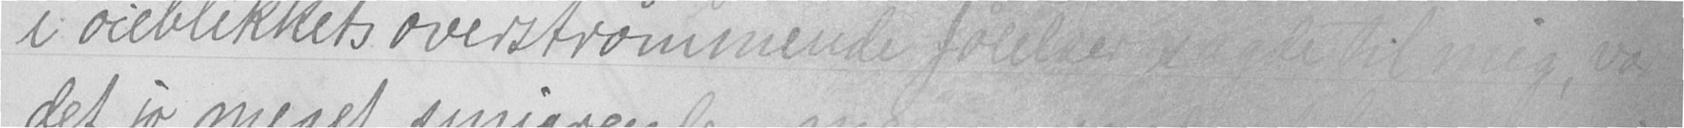i øieblikkets overstrømmende følelser sagde til mig, var
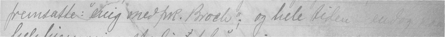fremsatte: "enig med frk. Broch"; og hele tiden - endog paa
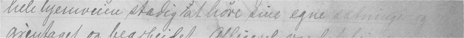hele hjemveien stadig at høre sine egne sætninger og ord
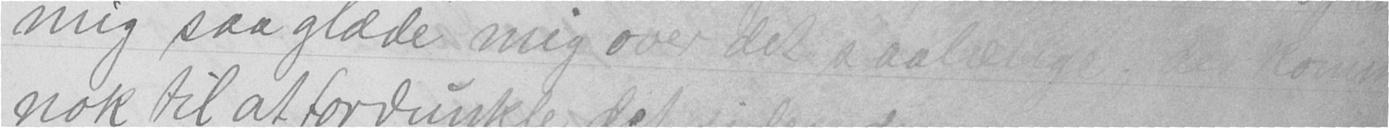mig saa glæde mig over det saalænge; det kommer
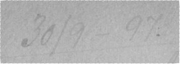30/9-97.
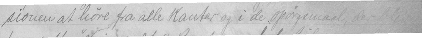sionen at høre fra alle kanter og i de spørgsmaal, der blev
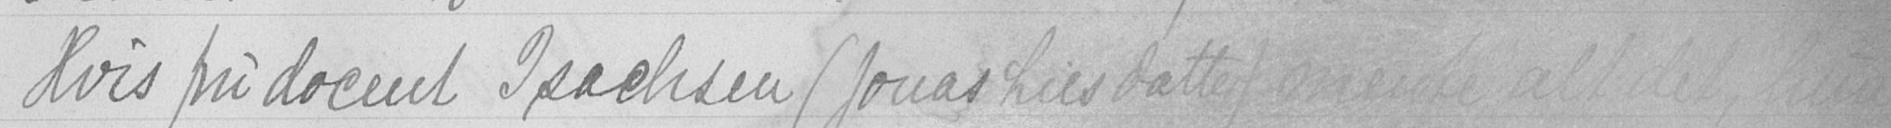Hvis fru docent Isachsen (Jonas Lies datter) mente alt det, hun
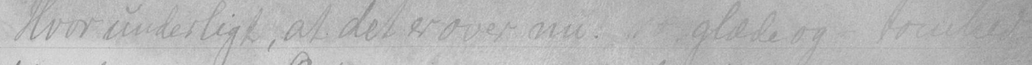Hvor underligt, at det er over nu.  glæde og tomhed
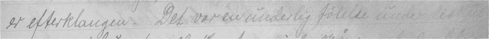er efterklangen. Det var en underlig følelse under diskus-
